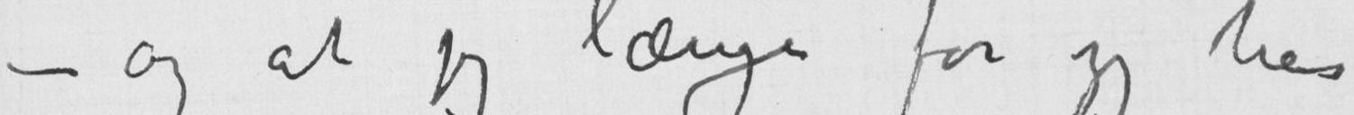– og at jeg længe før jeg har
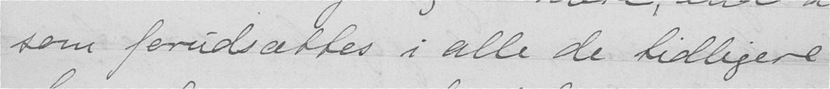som forudsættes i alle de tidligere
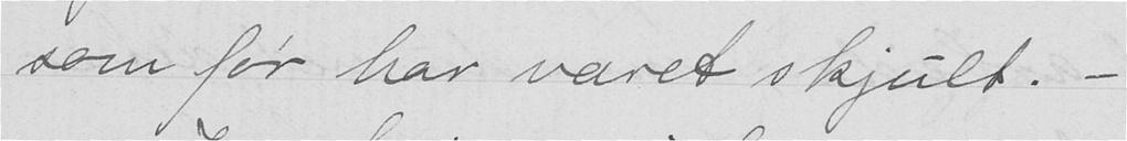som før har været skjult. -
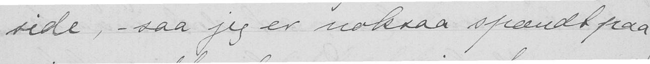side, - saa jeg er noksaa spendt paa
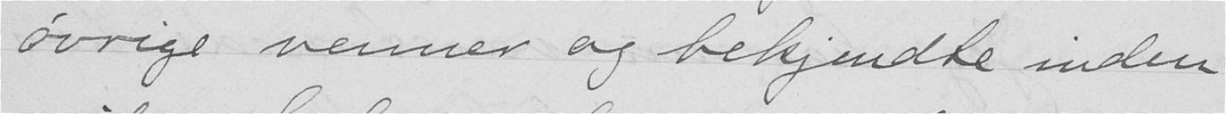øvrige venner og bekjendte inden
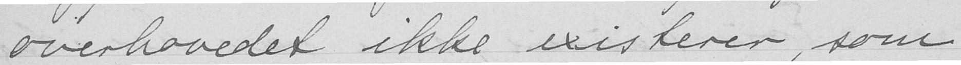overhovedet ikke existerer, som
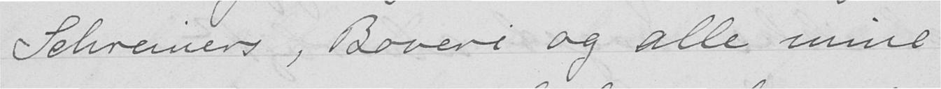Schreiners, Boveri og alle mine
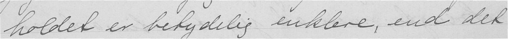holdet er betydelig enklere, end det
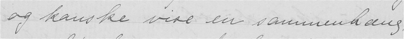og kanske vise en sammenhæng,
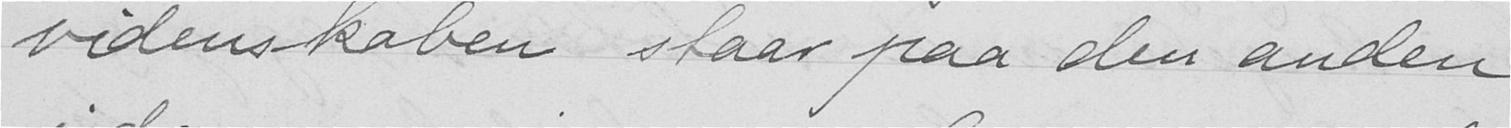videnskaben staar paa den anden
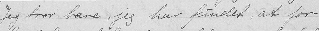Jeg tror bare, jeg har fundet, at for-
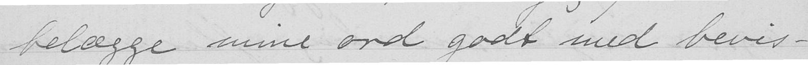belægge mine ord godt med bevis-
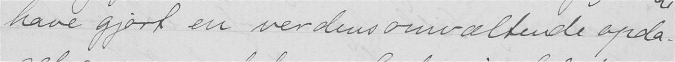have gjort en verdensomvæltende opda-
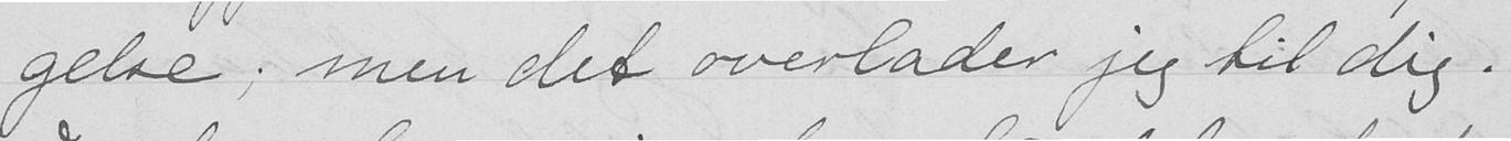gelse; men det overlader jeg til dig.
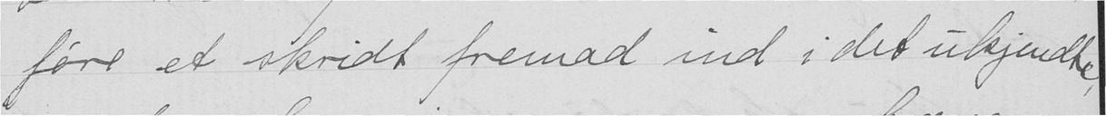føre et skridt fremad ind i det ukjendte,
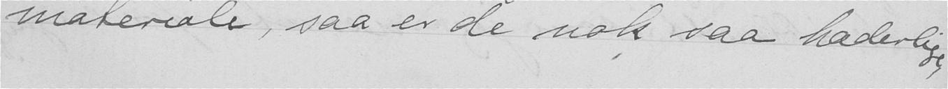materiale, saa er de nok saa hæderlige,
